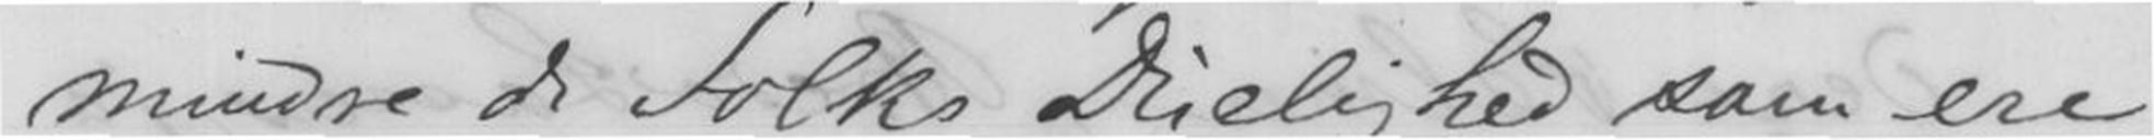mindre de Folks Duelighed som ere
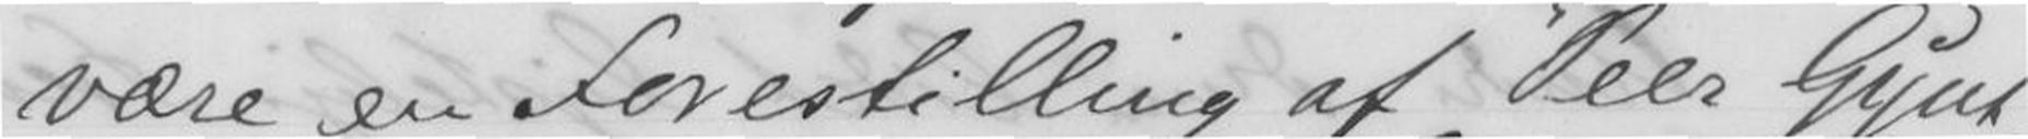være en Forestilling af "Peer Gynt"
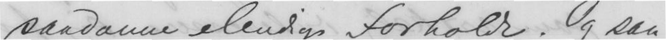saadanne elendige Forholde. Og saa
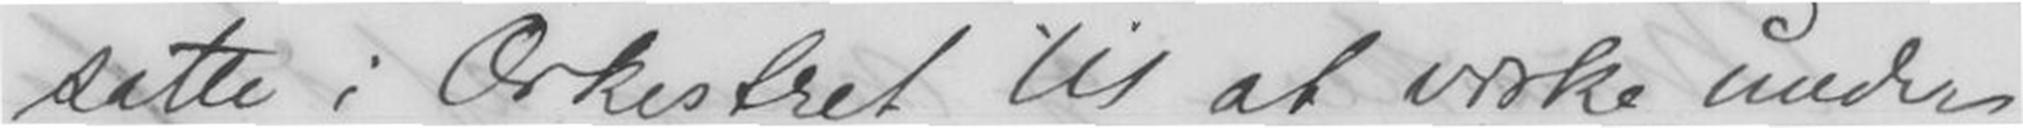satte i Orkestret til at virke undet
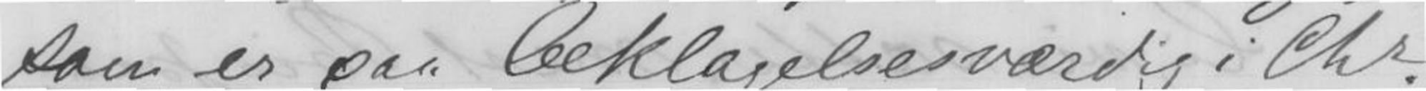som er saa beklagelsesværdig i Chr.,
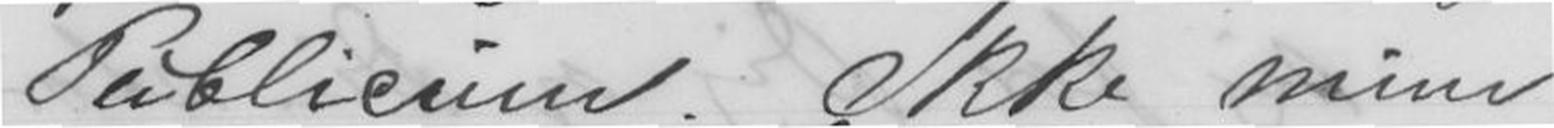Publikum. Ikke mine
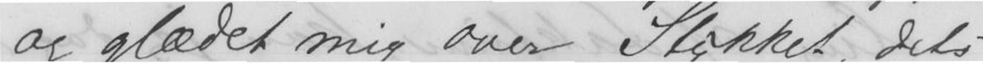og glædet mig over Stykket dets
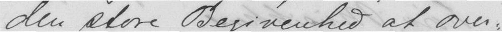den store Begivenhed at over-
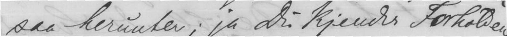saa herunter; ja Du kjender Forholdene,
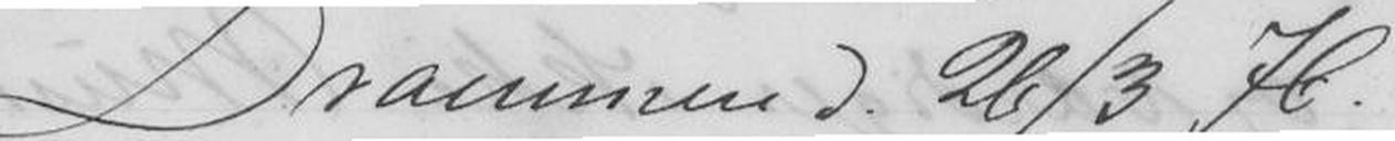Drammen d. 26/3 76
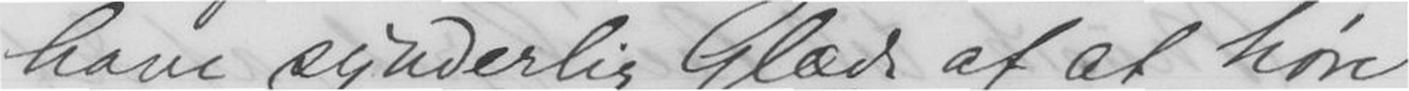have synderlig Glæde af at høre
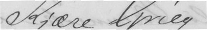Kjære Grieg
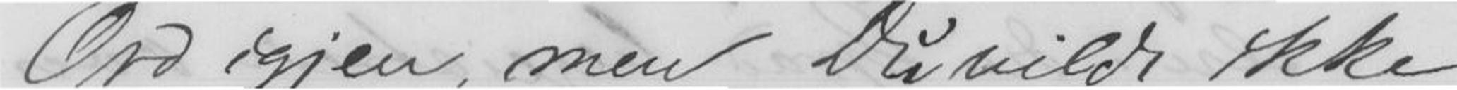Ord igjen, men Du vilde ikke
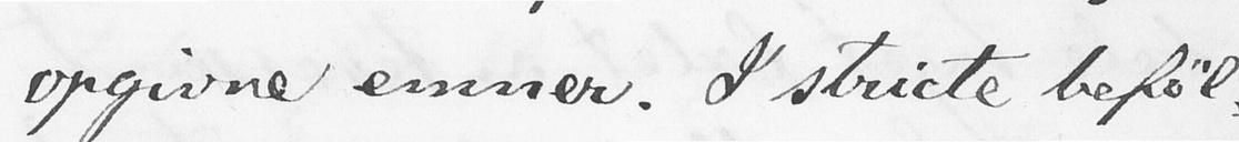opgivne emner. I stricte beføl-
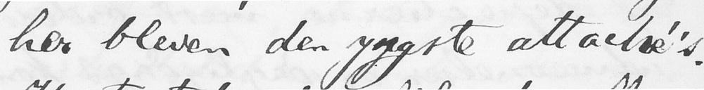her bleven den yngste attaché's.
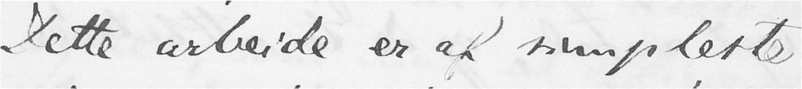Dette arbeide er af simpleste
